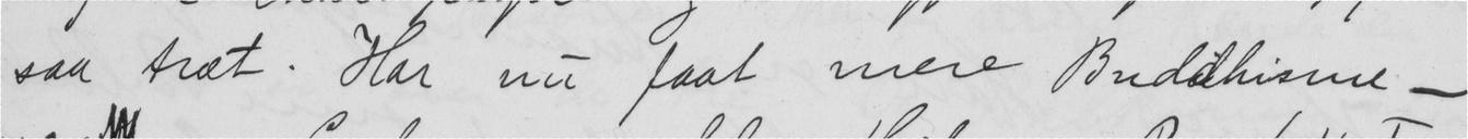saa træt. Har nu faat mere Buddhisme –
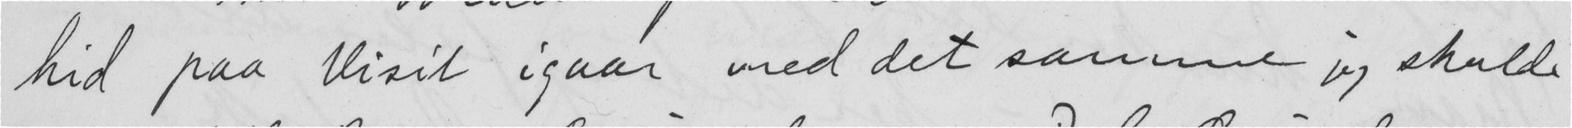hid paa Visit igaar med det samme jeg skulde
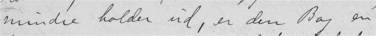mindre holder ud, er den Bog en
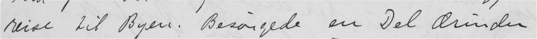reise til Byen. Besørgede en Del Ærinder
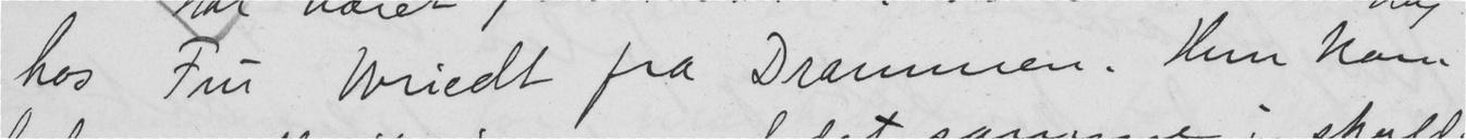hos Fru Wriedt fra Drammen. Hun kom
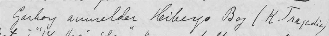Garborg anmelder Heibergs Bog (K. Tragedie)
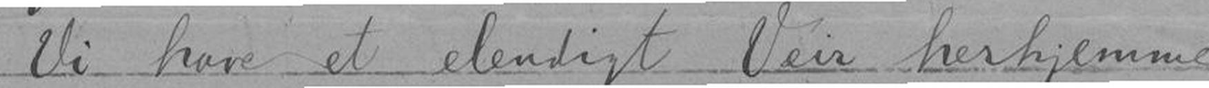Vi have et elendigt Veir herhjemme,
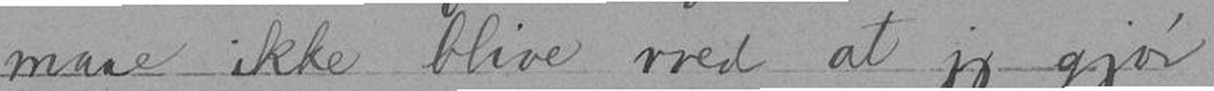maae ikke blive vred at jeg gjør
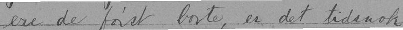ere de først borte, er det tidsnok
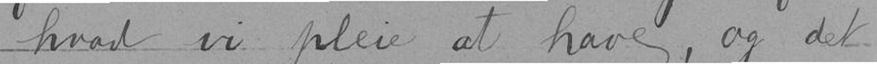hvad vi pleie at have, og det
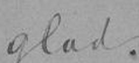glad.
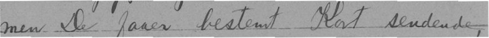men De faaer bestemt Kort sendende,
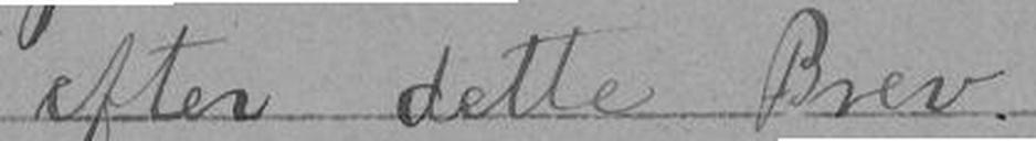efter dette Brev.
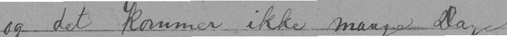og det kommer ikke mange Dage
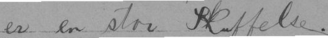er en stor Skuffelse.
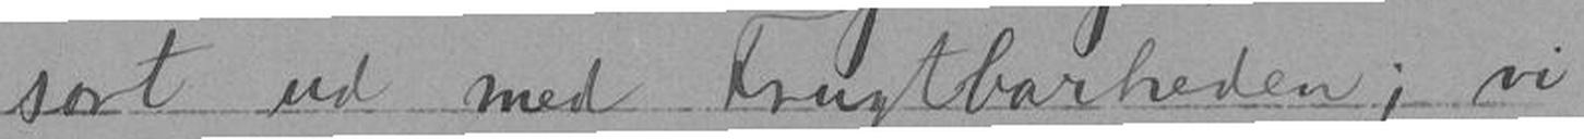sort ud med Frugtbarheden; vi
